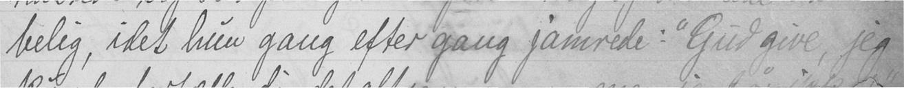belig, idet hun gang efter gang jamrede: "Gud give, jeg
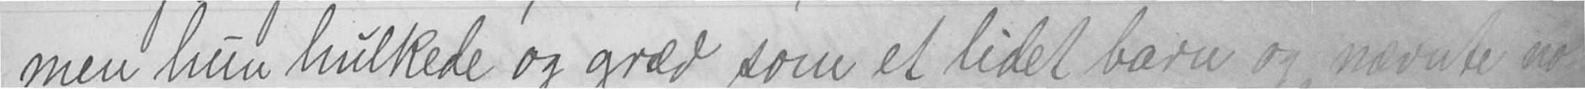men hun hulkede og græd som et lidet barn og nævnte no-
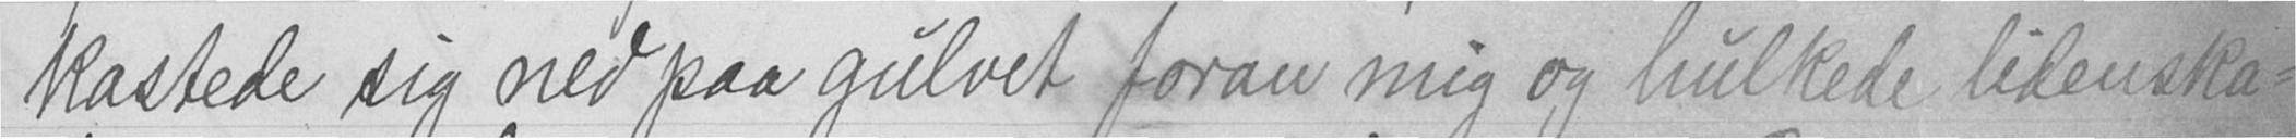kastede sig ned paa gulvet foran mig og hulkede lidenska-
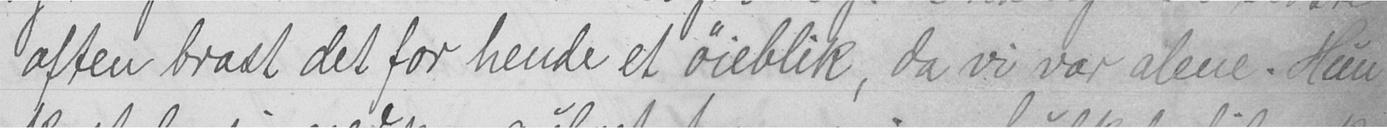aften brast det for hende et øieblik, da vi var alene. Hun
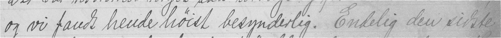og vi fandt hende høist besynderlig. Endelig den sidste
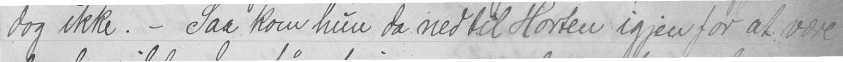dog ikke. - Saa kom hun da ned til Horten igjen for at være
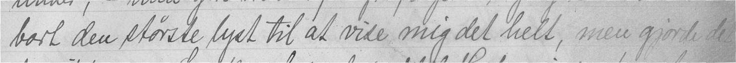bart den største lyst til at vise mig det helt, men gjorde det
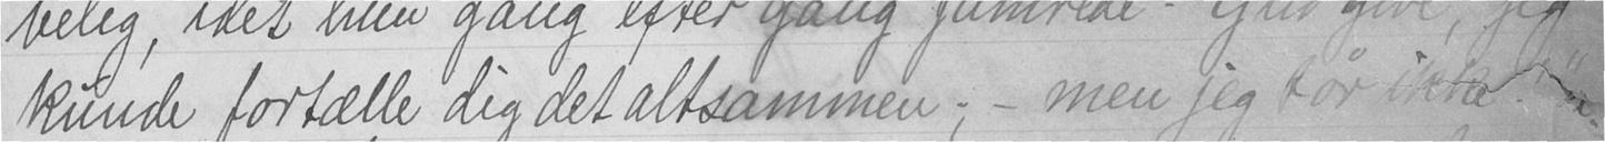kunde fortælle dig det altsammen; - men jeg tør ikke!"
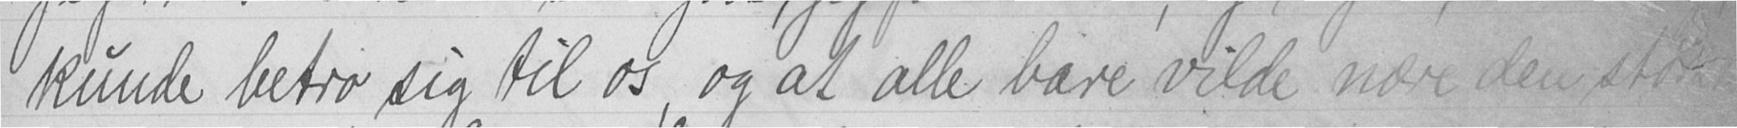kunde betro sig til os, og at alle bare vilde nære den store
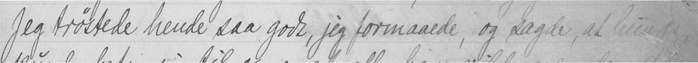Jeg trøstede hende saa godt, jeg formaaede, og sagde at hun
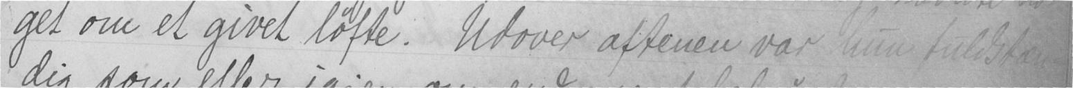get om et givet løfte. Udover aftenen var hun fuldsten-
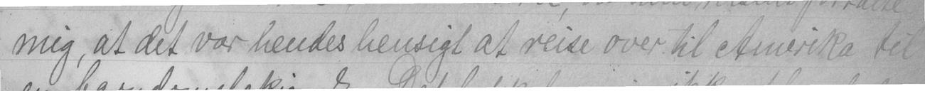mig, at det var hendes hensigt at reise over til Amerika til
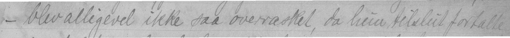- blev alligevel ikke saa overrasket, da hun tilslut fortalte
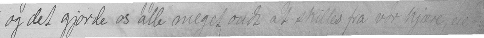og det gjorde os alle meget ondt at skilles fra vor kjære eie-
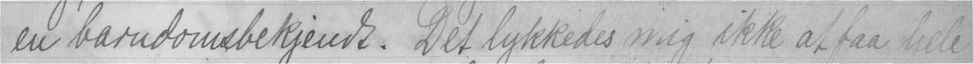en barndomsbekjendt. Det lykkedes mig ikke at faa hele
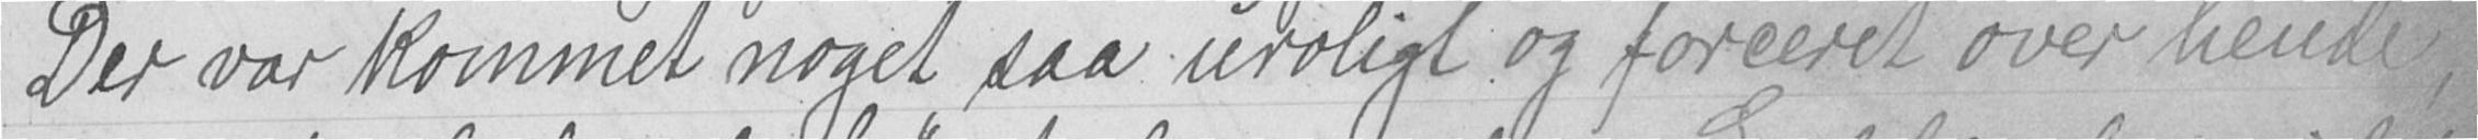Der var kommet noget saa uroligt og forceret over hende,
